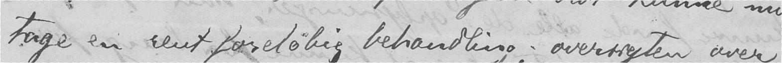tage en rent foreløbig behandling; oversigten over
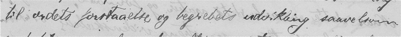til ordets forstaaelse og begrebets udvikling saavelsom
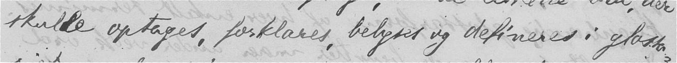skulde optages, forklares, belyses og defineres i glossa-
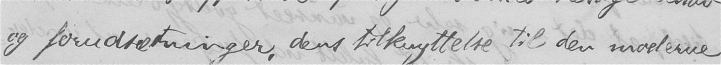og forudsætninger, dens tilknyttelse til den moderne
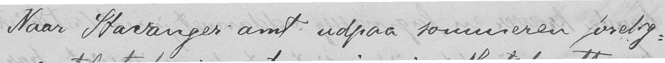Naar Stavanger amt udpaa sommeren forelig-
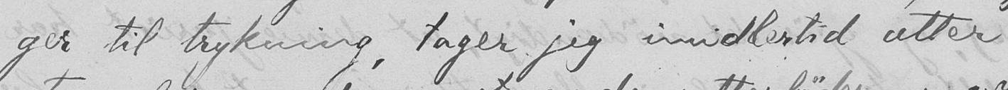ger til trykning, tager jeg imidlertid atter
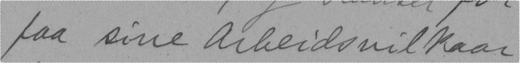faa sine Arbeidsvilkaar
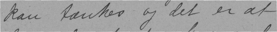kan tænkes og det er at
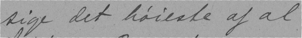sige det høieste af al
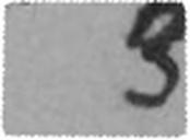3
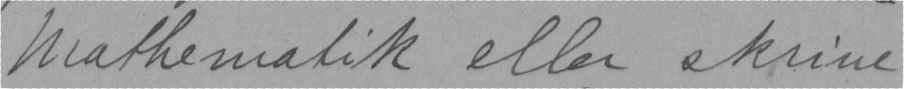mathematik eller skrive
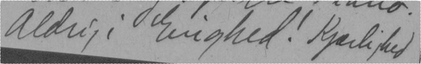Aldrig i Evighed! Kjærliged
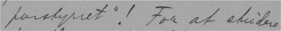forstyrret"! For at studere
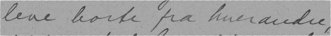leve borte fra hverandre,
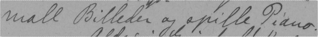male Billeder og spille Piano.
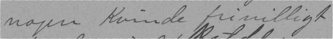nogen Kvinde frivilligt
